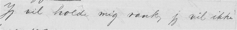Jeg vil holde mig rank, jeg vil ikke
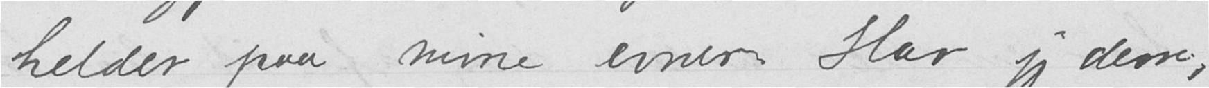helder paa mine evner. Har jeg dem,
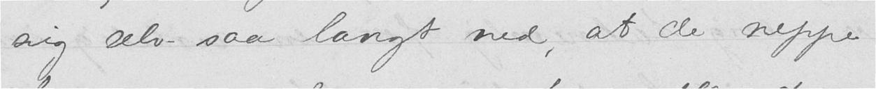sig selv saa langt ned, at de neppe
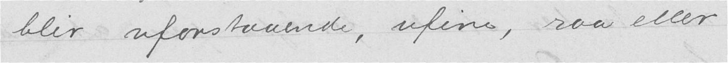blir uforstaaende, ufine, raa eller
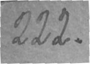222.
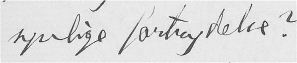synlige fortrydelse?
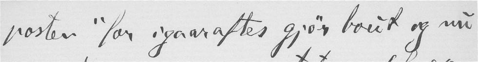posten" for igaaraftes gjør bout og nu
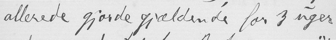allerede gjorde gjældende for 3 uger
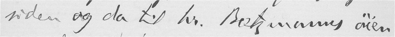siden og da til hr. Bætzmanns øien-
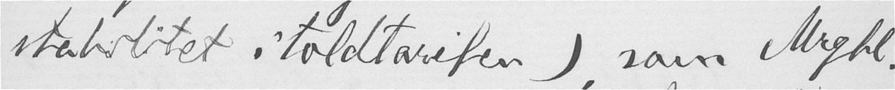stabilitet i toldtarifen), som Mrgbl.
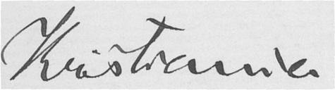Kristiania
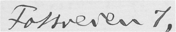Fossveien 7,
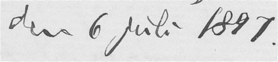den 6 juli 1897.
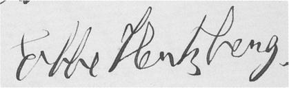Ebbe Hertzberg.
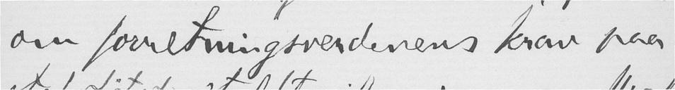om forretningsverdenens krav paa
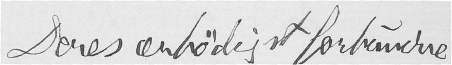Deres ærbødigst forbundne
